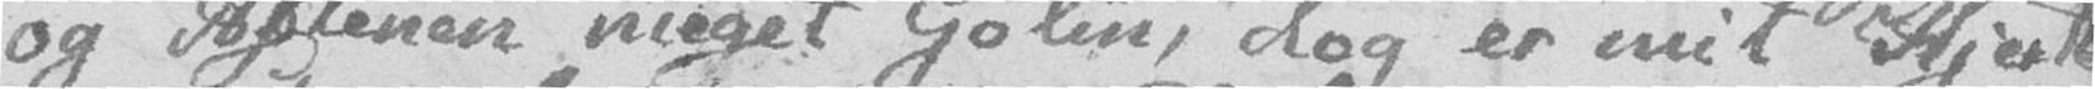og Aftenen meget Golin, dog er mit Hjerte
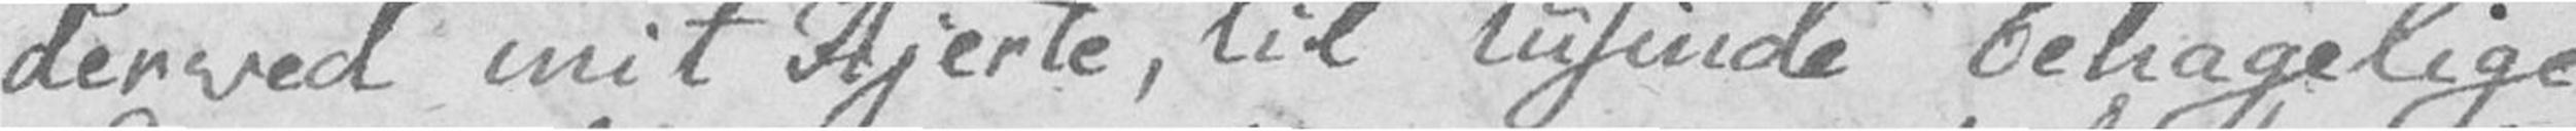derved mit Hjerte, til tusende behagelige
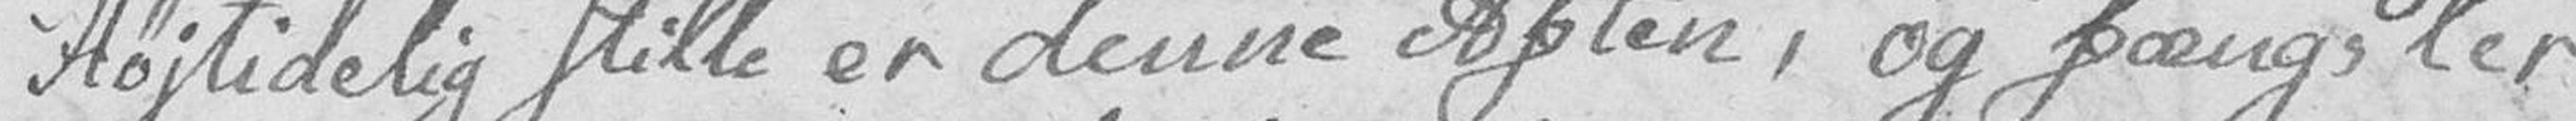Højtidelig stille er denne Aften, og fængsler
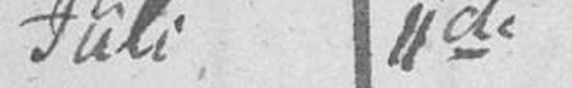Juli 11de
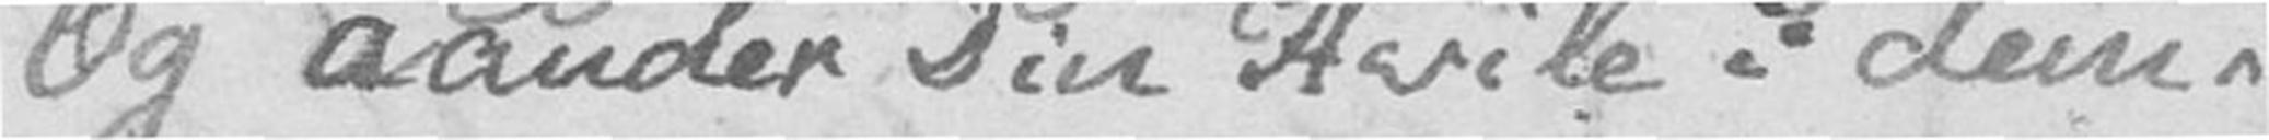Og aanden Din Hvile i dem.
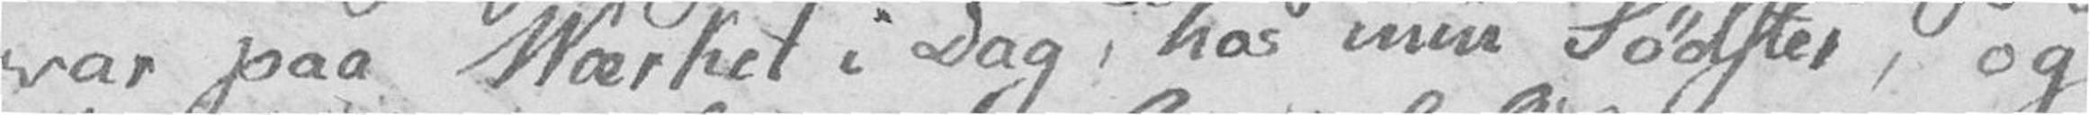var paa Værket i Dag, hos min Sødster, og
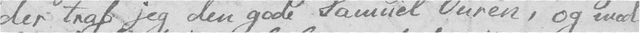der traf jeg den gode Samuel Ouren, og med
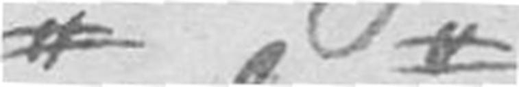# #
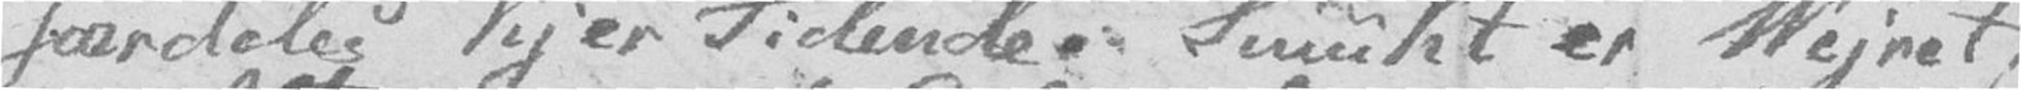særdeles kjer Tidende. Smukt er Vejret,
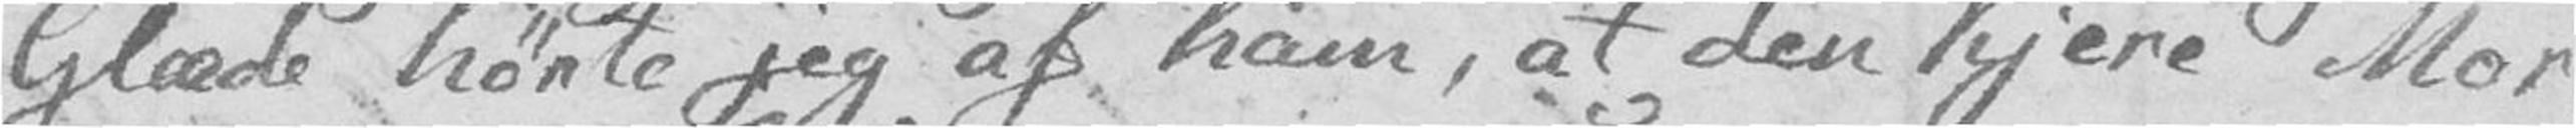Glæde hørte jeg af ham, at den kjere Mor
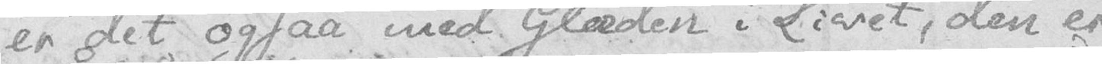er det ogsaa med Glæden i Livet, den er
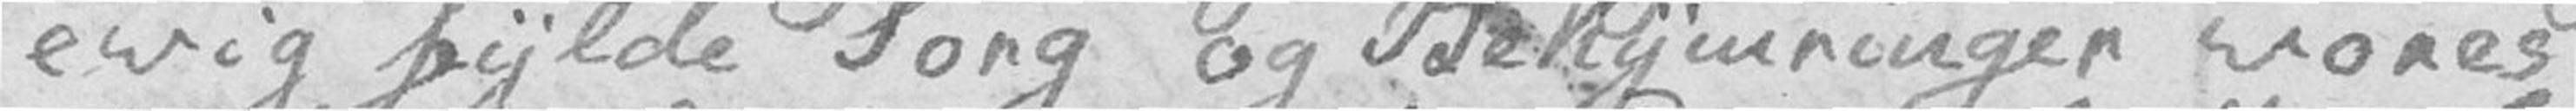evig fylde Sorg og Bekymringer vores
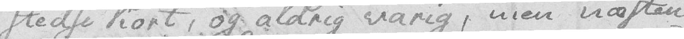stedse kort, og aldrig varig, men næsten
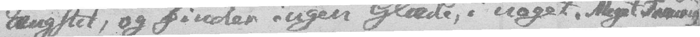ængstet, og finder ingen Glæde, i noget. Meget Traurig
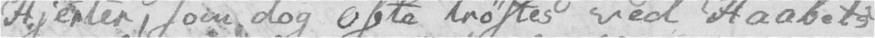Hjerte, som dog ofte trøstes ved Haabets
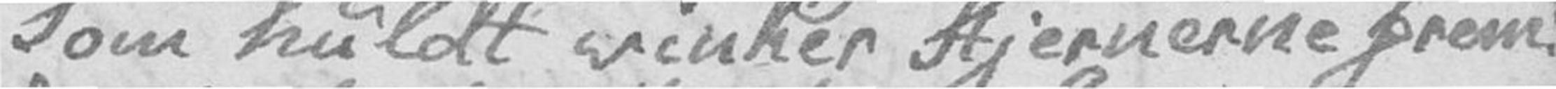Som huldt vinker Stjernerne frem
